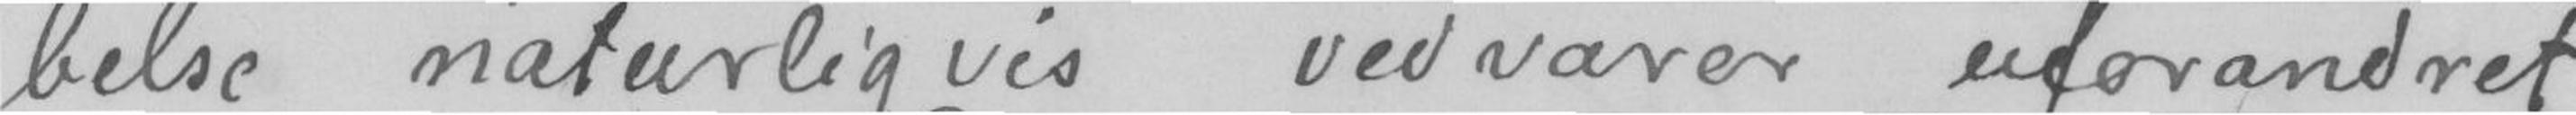belse naturligvis vedvarer udforandret
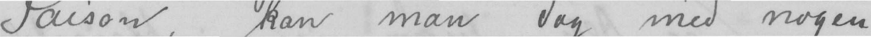Paison, kan man dog med nogen
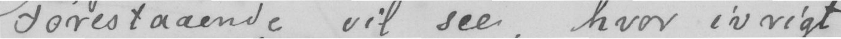Forestaaende vil see, hvor ivrigt
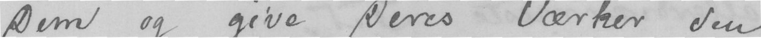Dem og give Deres Værker den
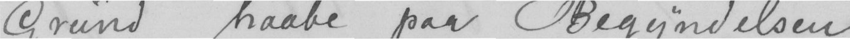Grund haabe paa Begyndelsen
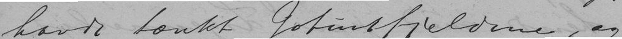havde tænkt Jotunfjeldene, og
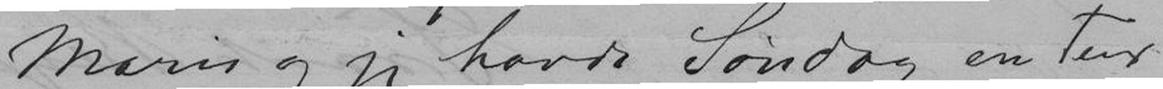Marie og jeg havde Søndag en Tur
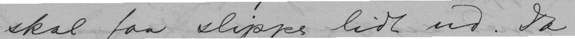jeg skal faa slippe lidt ud. Da
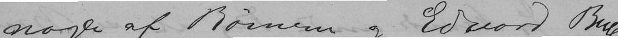nogle af Børnene og Edvard Bull.
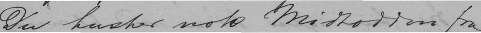Du husker nok Midtodden fra
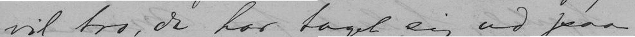vil tro, de har taget sig ud paa
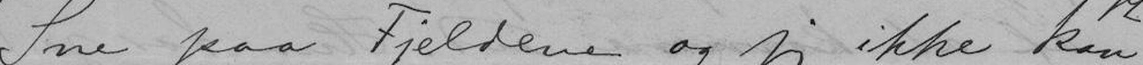Sne paa Fjeldene og jeg ikke kan
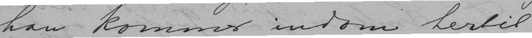han kommer indom hertil.
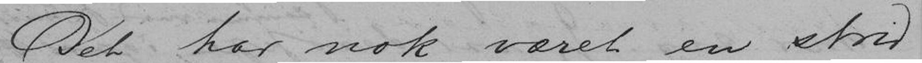Det har nok været en strid
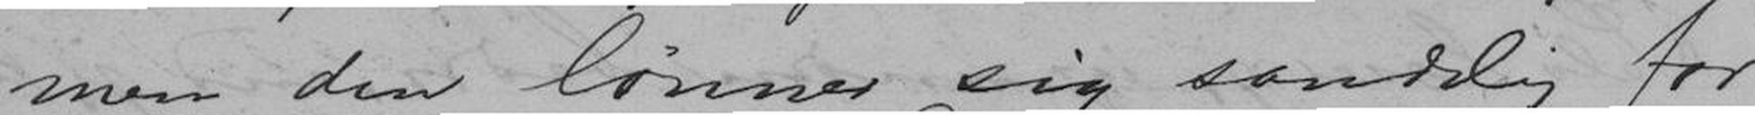men den lønner sig sandelig for
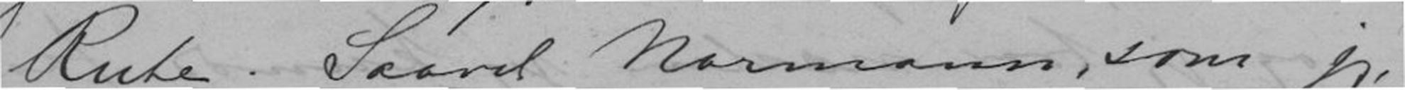Rute. Saavel Normann, som jeg,
